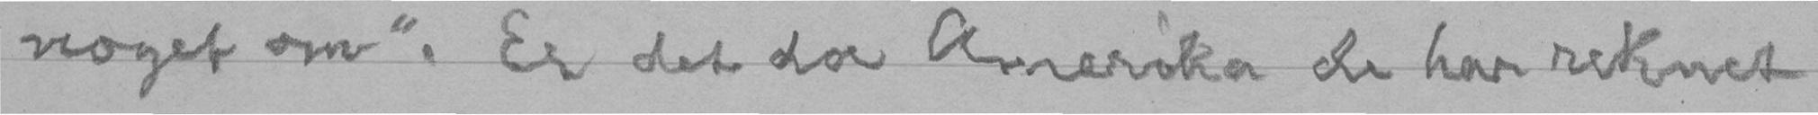noget om". Er det da Amerika de har reknet
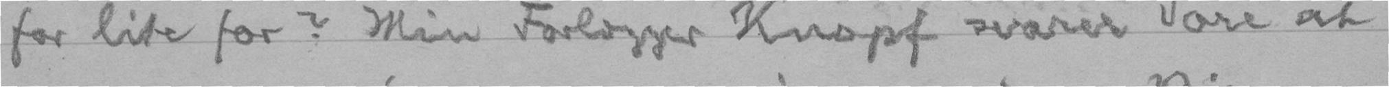for lite for? Min Forlægger Knopf svarer Tore at
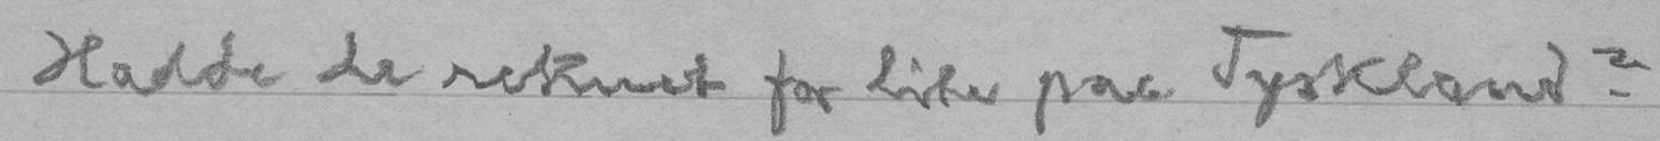Hadde de reknet for lite paa Tyskland?
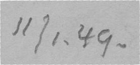11/1. 49.
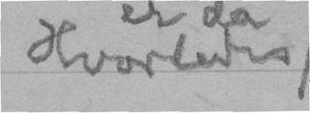Hvorledes
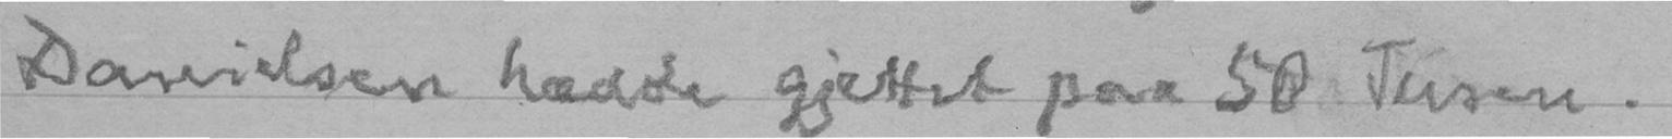Danielsen hadde gjettet paa 50 Tusen.
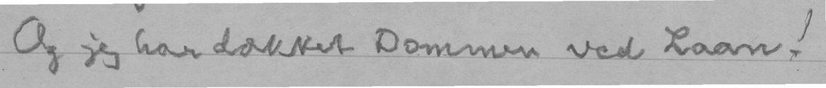Og jeg har dækket Dommen ved Laan!
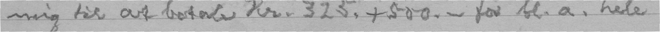mig til at betale Kr. 325. + 500. - for bl.a. hele
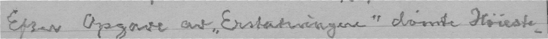Efter Opgave av "Erstatningen" dømte Høieste.
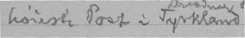høieste Post i Tyskland
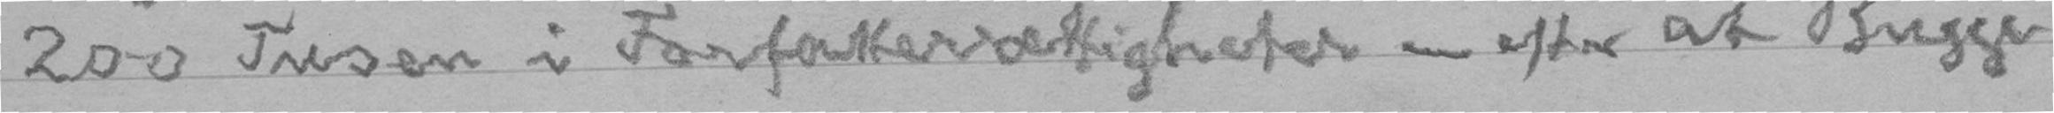200 Tusen i Forfatterrættigheter - efter at Bugge
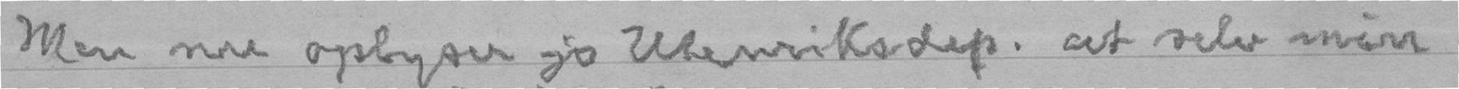Men nu oplyser jo Utenriksdep. at selv min
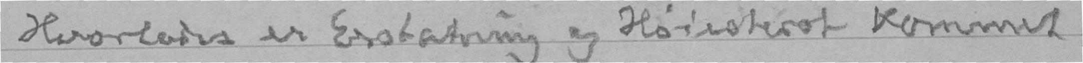Hvorledes er Erstatning og Høiesterot kommet
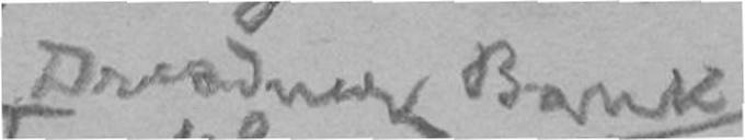Dresdner Bank
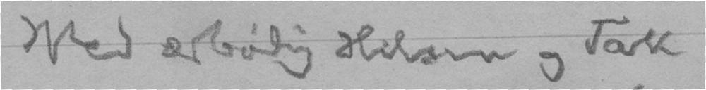Med ærbødig Hilsen og Tak
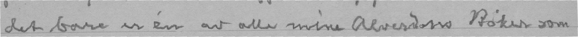det bare er én av alle mine Alverdens Bøker som
